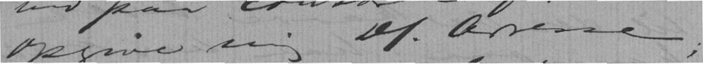opgive mig Ds. Adresse;
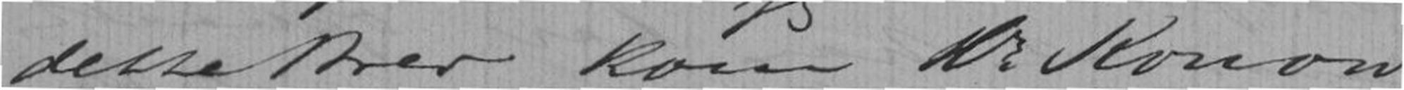dette Brev kom Hr. Konow
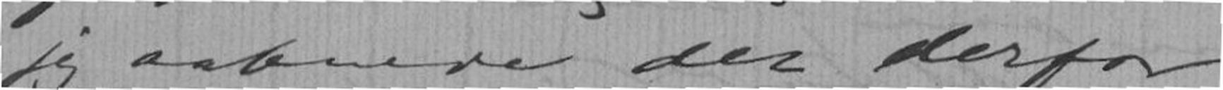jeg aabnede det derfor
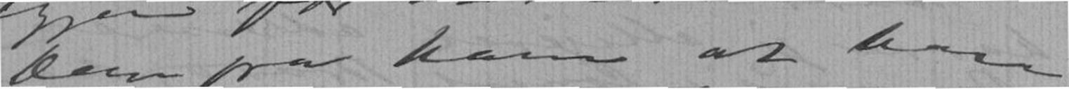Dem fra ham at han
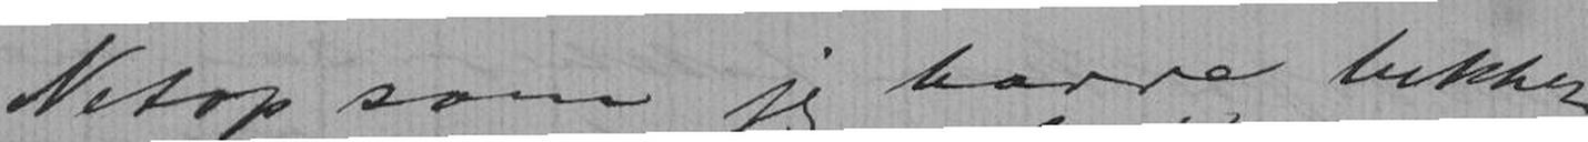Netop som jeg havde lukket
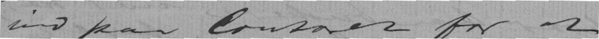ind paa Contoret for at
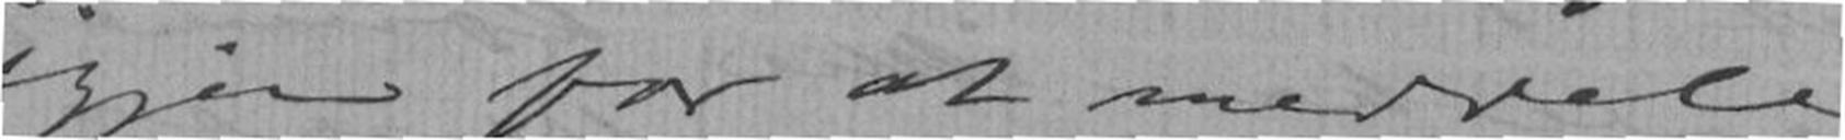igjen for at meddele
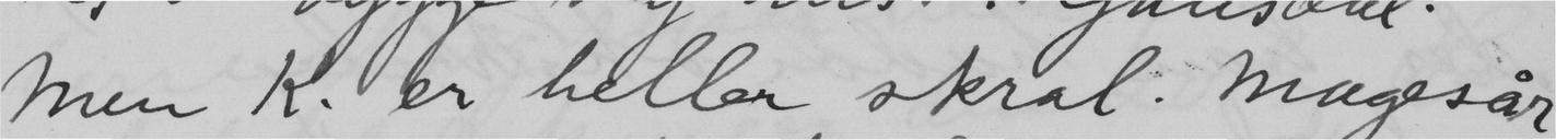Men K. er heller skral. Magesår
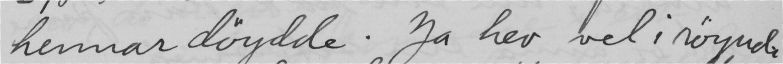hennar döydde. Ja hev vel i röynde
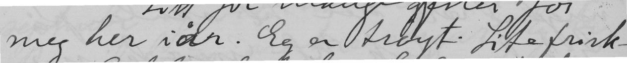meg her iår. Eg er tröyt. Lite frisk –
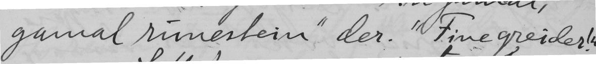gamal runestein" der. "Fine greider!"
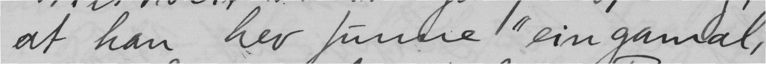at han hev funne "ein gamal,
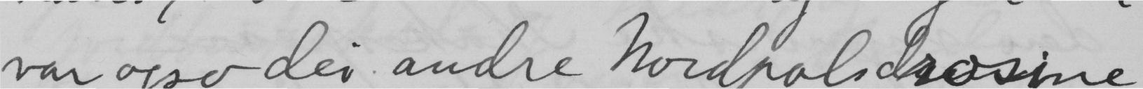var ogso dei andre Nordpolsdrosine
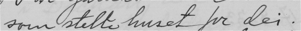som stelte huset for dei.
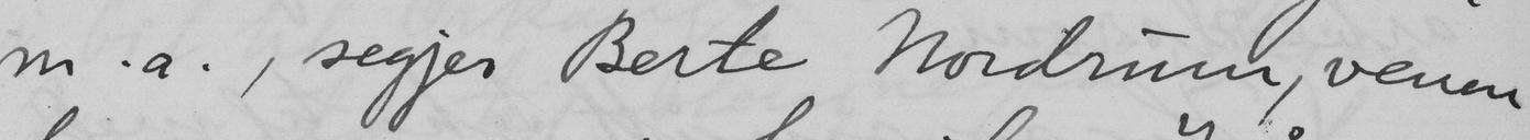m.a., segjer Berte Nordrum, venen
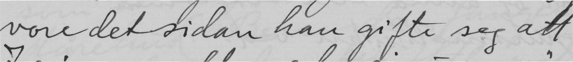vore det sidan han gifte seg att
